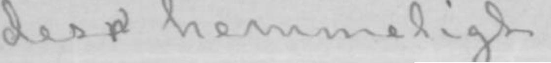des hemmeligt!
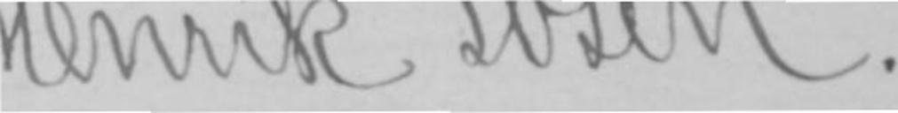Henrik Ibsen.
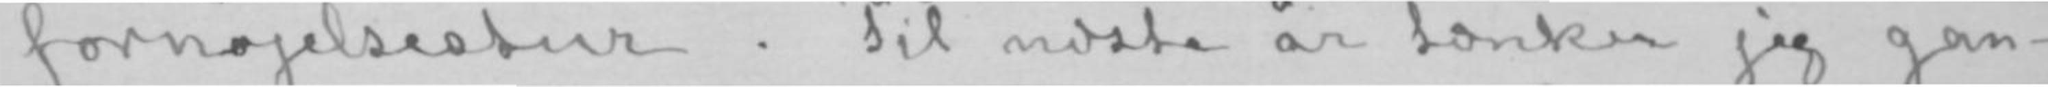fornøjelsestur. Til næste år tænker jeg gan-
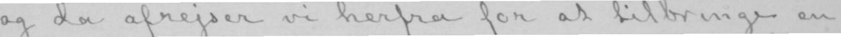og da afrejser vi herfra for at tilbringe en
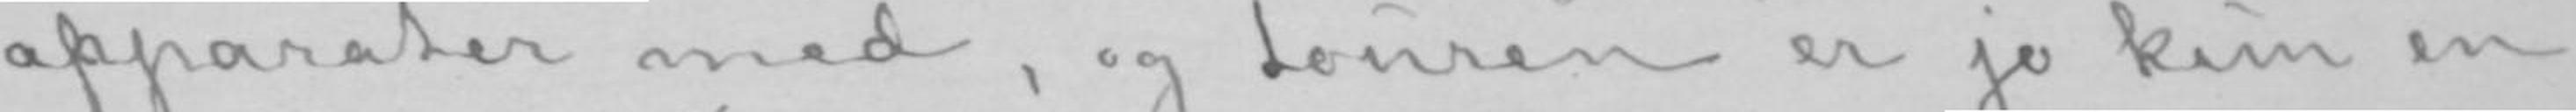apparater med, og touren er jo kun en
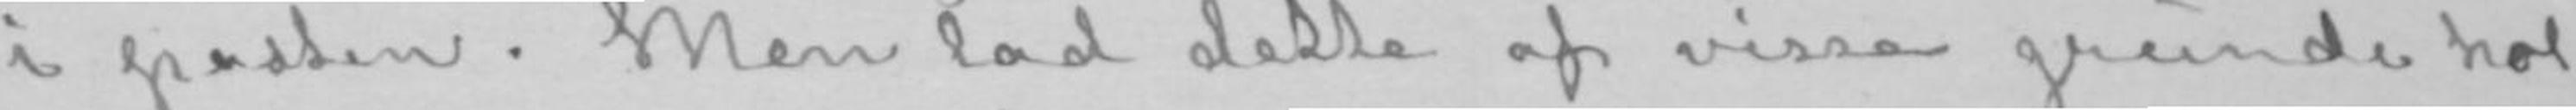i posten. Men lad dette af visse grunde hol-
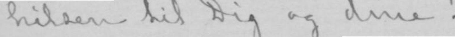hilsen til Dig og dine!
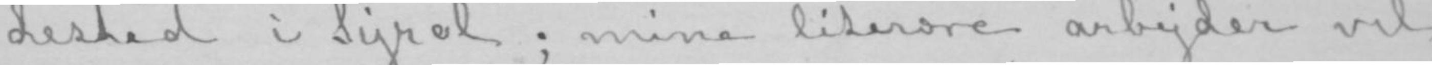dested i Tyrol; mine literære arbejder vil
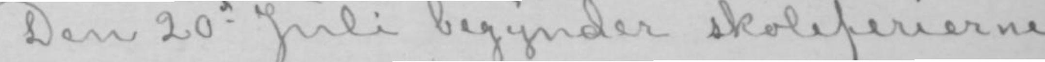Den 20de Juli begynder skoleferierne
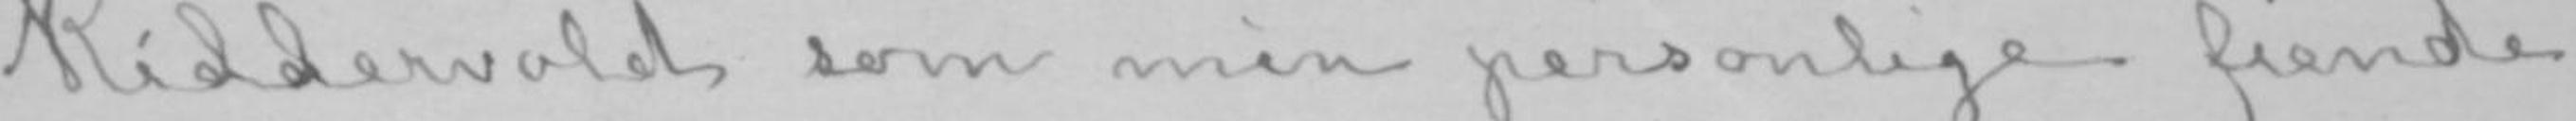Riddervold som min personlige fiende,
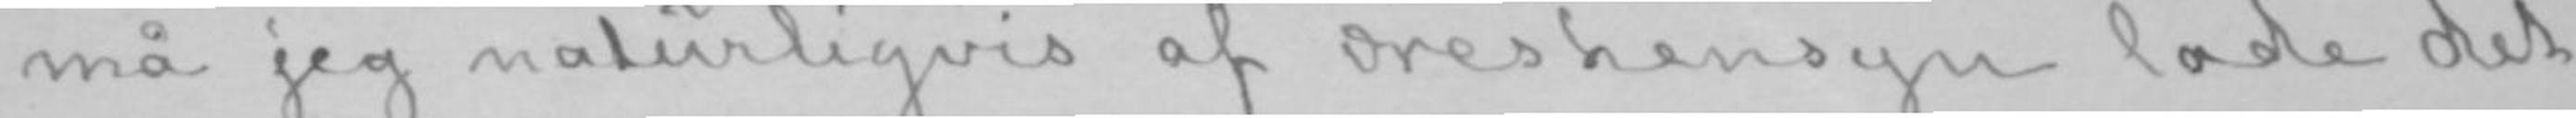må jeg naturligvis af æreshensyn lade det
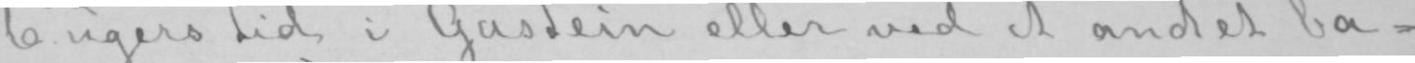6 ugers tid i Gastein eller ved et andet ba-
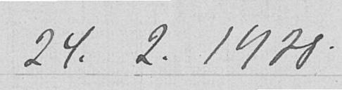24.2.1928.
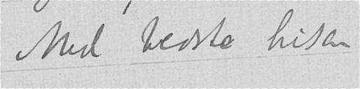Med bedste hilsen
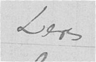Deres
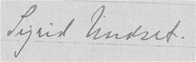Sigrid Undset.
